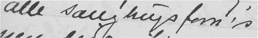alle saugbrugsforn's
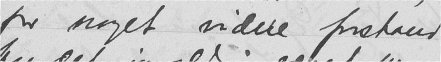for noget videre forstand
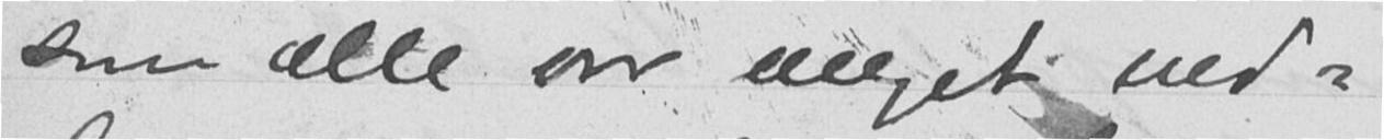som alle var meget ned-
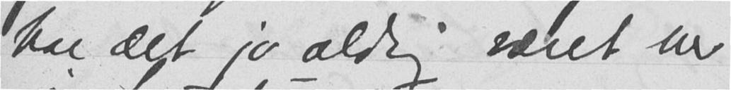har det jo aldrig været her
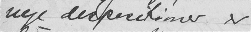nye dispositioner er
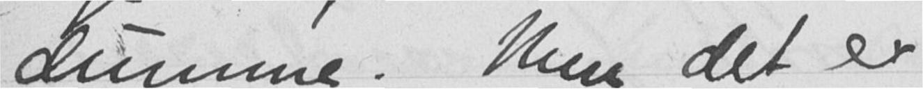dumme. "Men det er
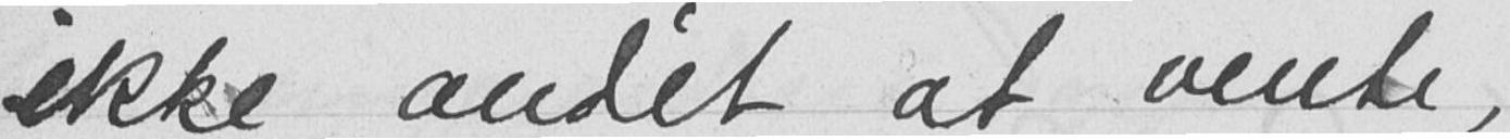ikke andet at vente,
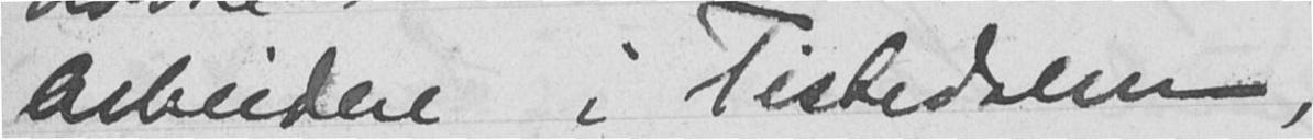arbeidere i Tistedalen,
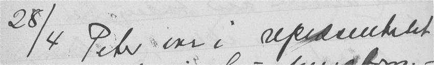28/4 Peter var i repræsentats-
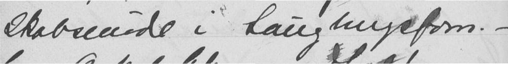skabsmøde i Saugbrugsforn. -
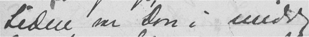Siden var Don i middag
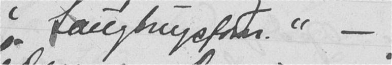i Saugbrugsforn." -
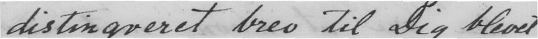distingveret brev til Dig blevet
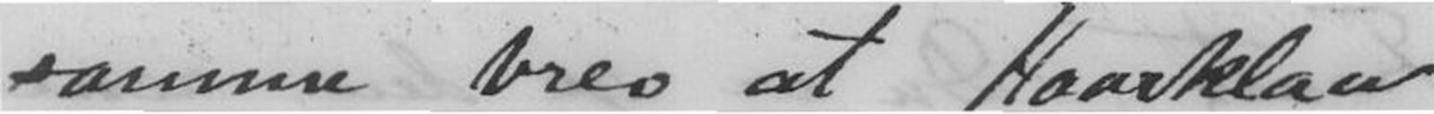samme brev at Haarklou
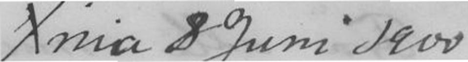Xnia, 8 Juni 1900
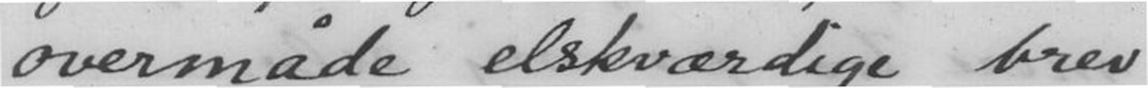overmåde elskværdige brev
